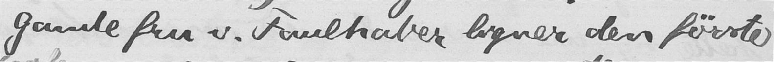Gamle fru v. Faulhaber ligner den første
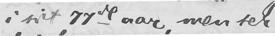i sit 77de aar, men ser
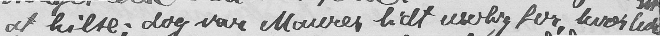at hilse; dog var Maurer lidt urolig for, hvorledes
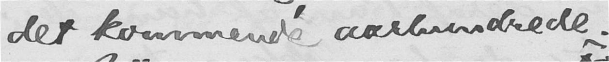det kommende aarhundrede.
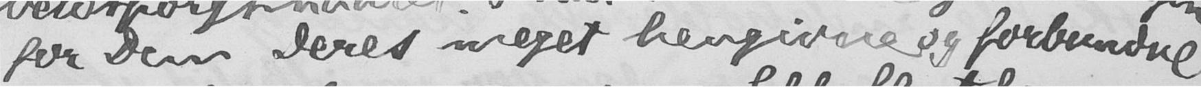for Dem Deres meget hengivne og forbundne
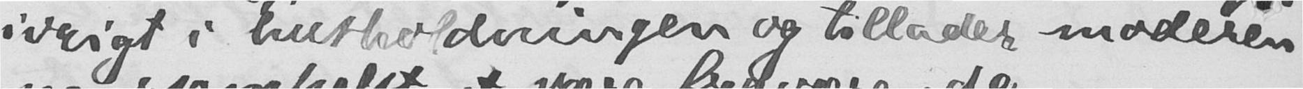ivrigt i husholdningen og tillader moderen
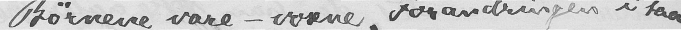Børnene vare - voxne. Forandringen i saa
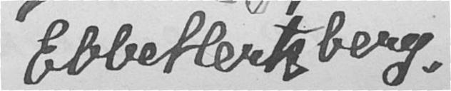Ebbe Hertzberg.
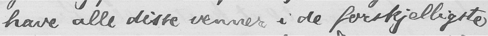have alle disse venner i de forskjelligste
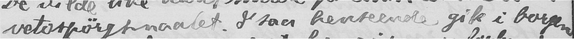vetospørgsmaalet. I saa henseende gik i borgen
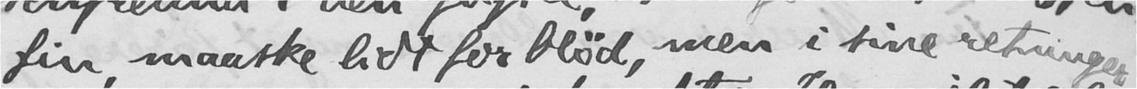fin, maaske lidt for blød, men i sine retninger
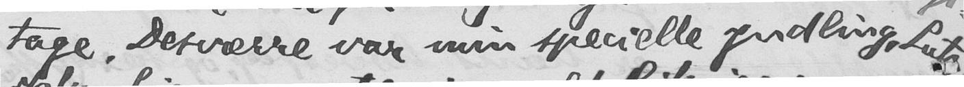tage. Desværre var min specielle yndling, Lutz,
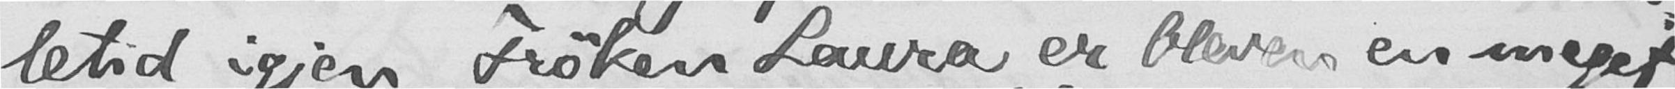letid igjen. Frøken Laura er bleven en meget
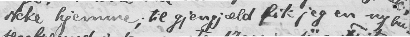ikke hjemme; til gjengjæld fik jeg en ny bu-
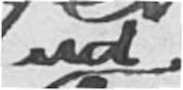ud
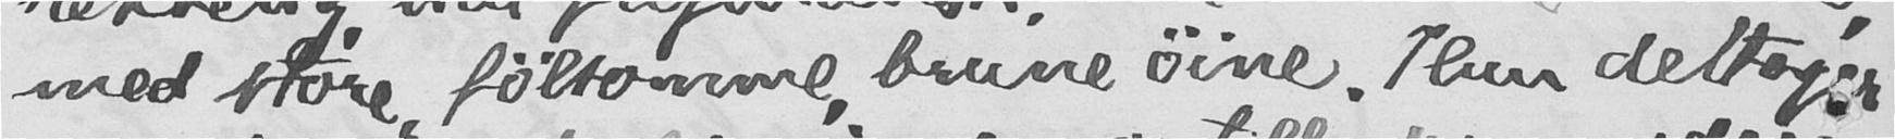med store, følsomme, brune øine. Hun deltager
